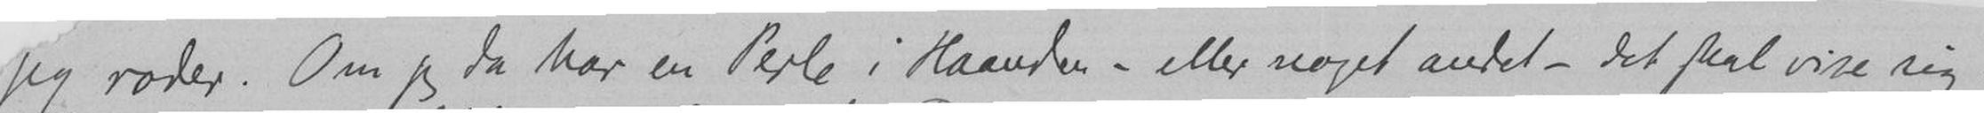jeg roder. Om jeg da har en Perle i Haanden - eller noget andet - det skal vise sig.
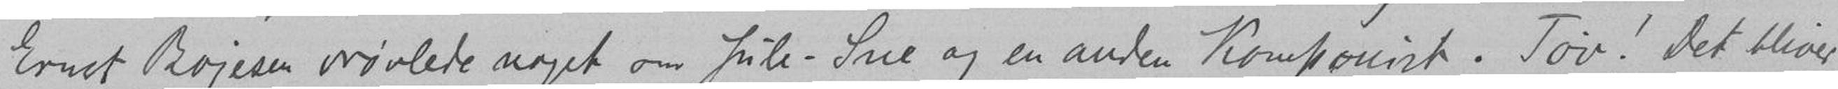Ernst Bojesen vrøvlede noget om Juli-Sne og en anden Komponist. Tøv! Det bliver
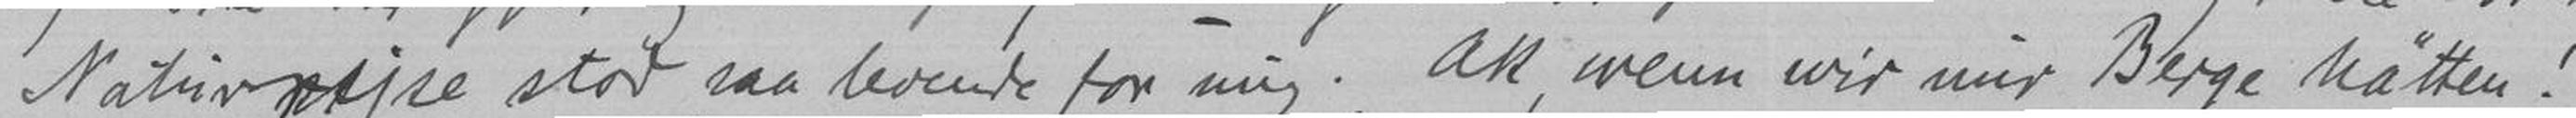Naturrejse stod saa levende for mig. Ah, wenn wir nur Berge Nätten"!
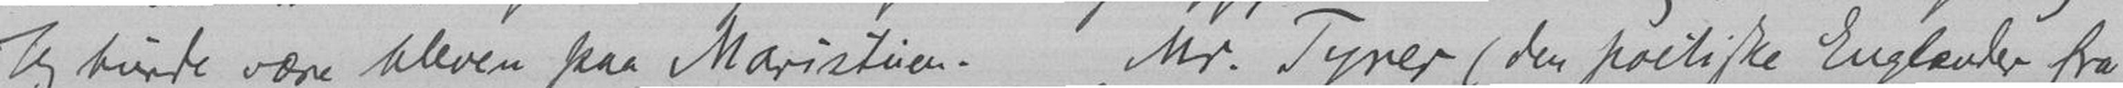Jeg burde være bleven paa Maristuen.Mr. Tyrer (den poitiske Englænder fra
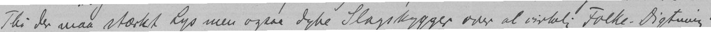Thi der maa strækt Lys men ogsaa dybe Slagskygger over al virkelig Folke-Digtning.
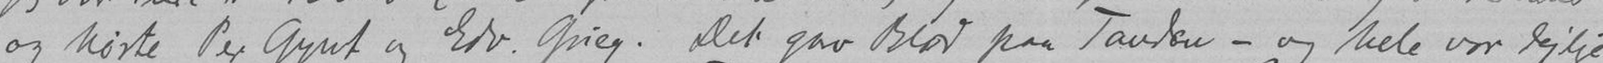og hørte Per Gynt og Edv. Grieg. Det gav Blod paa Tanden - og hele vor dejlige
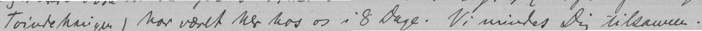Tvindehaugen) har været her hos os i 8 Dage. Vi mindes Dig tilsammen.
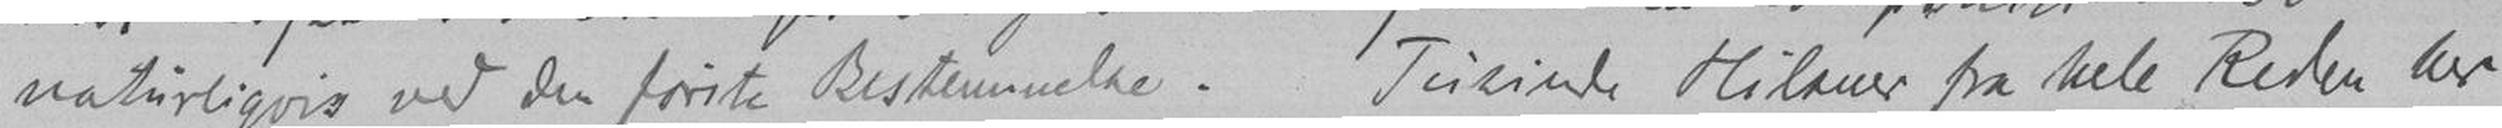naturligvis ved den første Bestemmelse.Tusind Hilsener fra hele Reden her
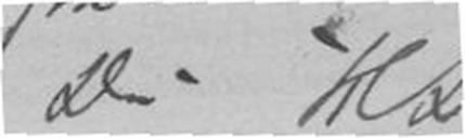Din HD.
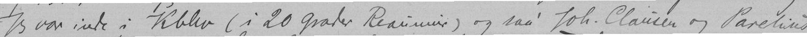Jeg var inde i Kbhv (i 20 Grader Reaumur) og saa Joh. Clausen og Parelius
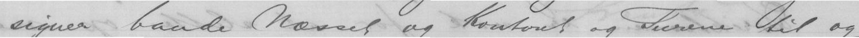signer baade Næsset og kontoret og Turene til og
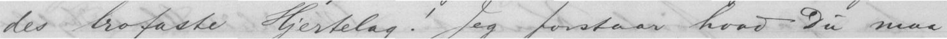des trofaste Hjertelag! Jeg forstaar hvad Du maa
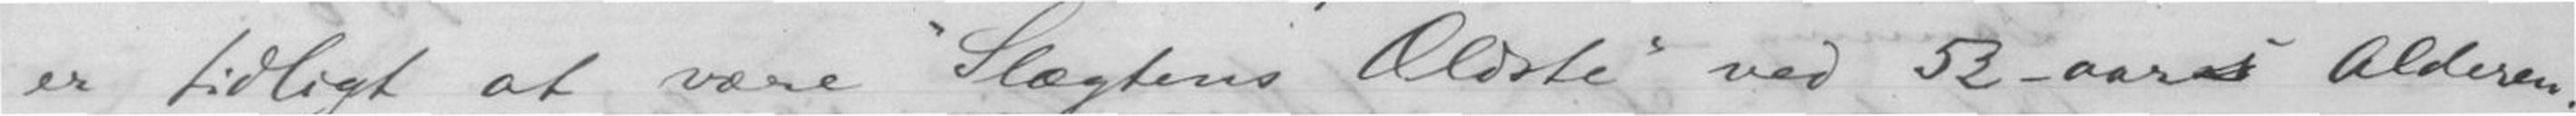er tidligt at være "Slægtens Ældste" ved 53-aars Alderen.
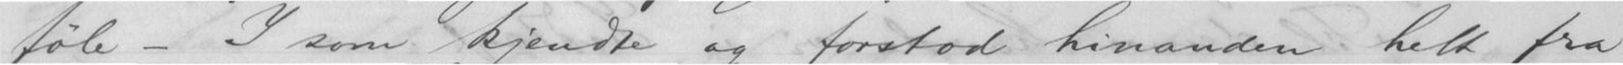føle - I som kjendte og forstod hinanden helt fra
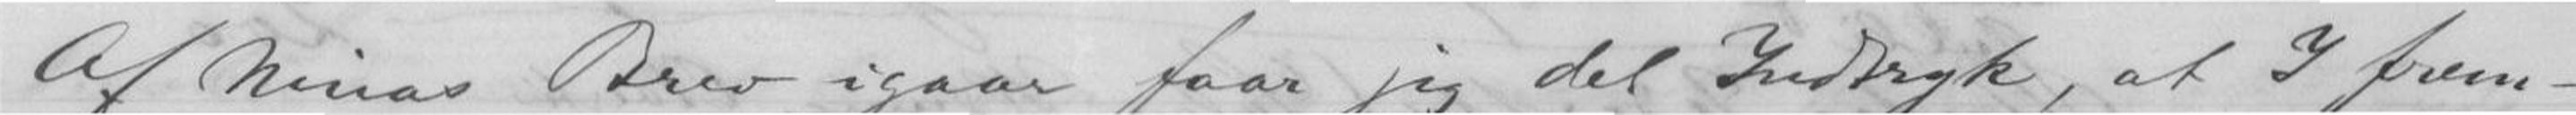Af Ninas Brev igaar faar jeg det Indtryk, at I frem-
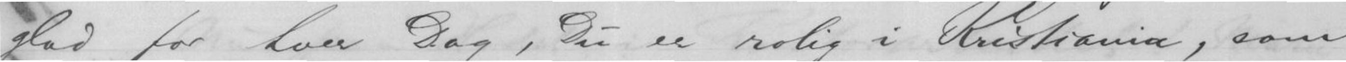glad for hver Dag, Du er rolig i Kristiania, som
